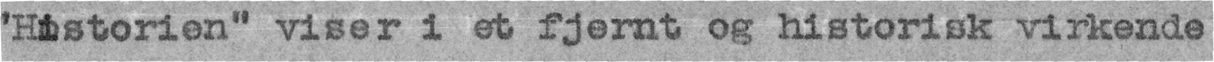«Hoistorien» viser i et fjernt og historisk virkende
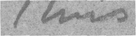Thiis
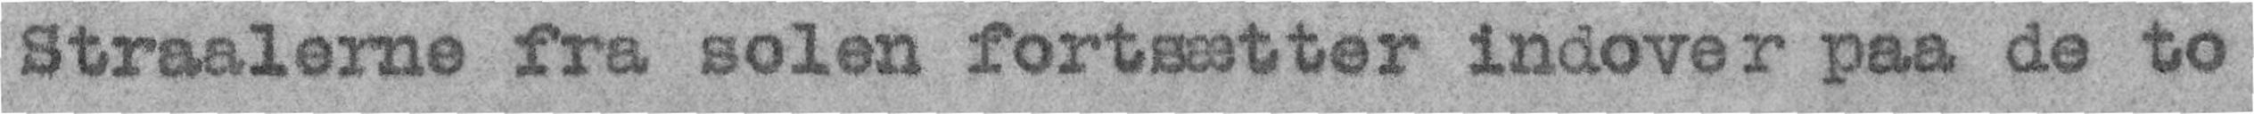Straalerne fra solen fortsætter indover paa de to
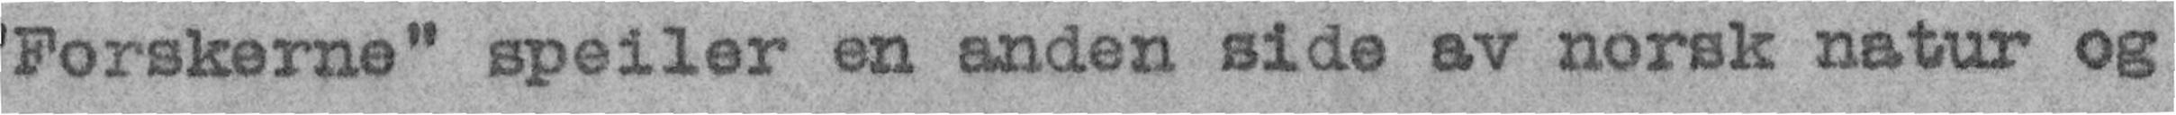«Forskerne» speiler en anden side av norsk natur og
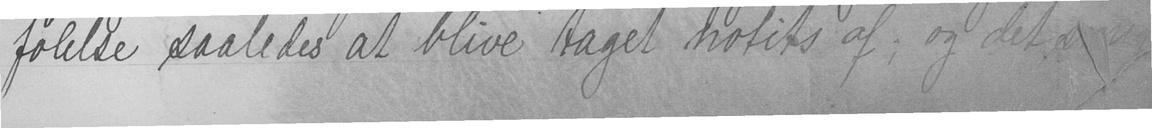følelse saaledes at blive taget notits af; og det s-
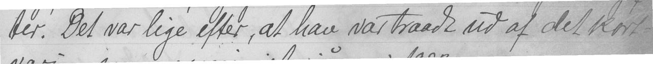ter. Det var lige efter, at han var traadt ud af det kort-
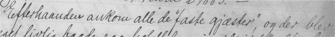Efterhaanden ankom alle de "faste gjæster", og der blev
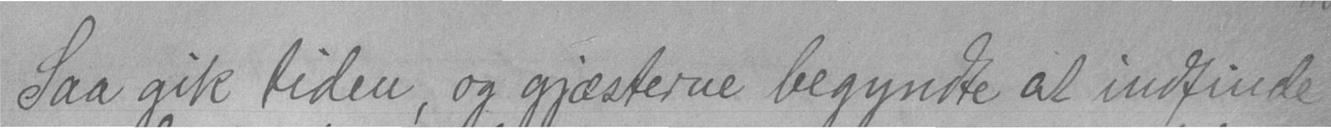Saa gik tiden, og gjæsterne begyndte at indfinde
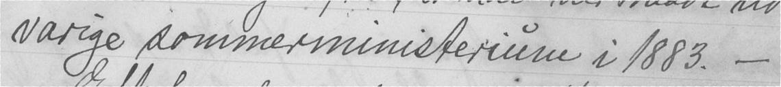varige sommerministerium i 1883. -
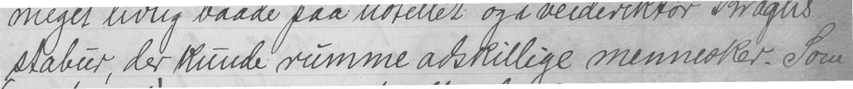stabur, der kunde rumme adskillige mennesker. Som
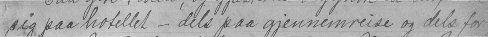sig paa hotellet - dels paa gjennemreise og dels for
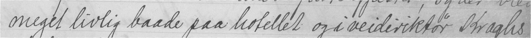meget livlig baade paa hotellet og i veidiriktør Kraghs
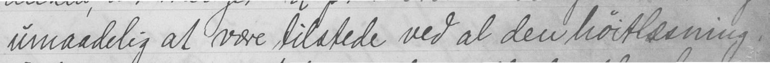umaadelig at være tilstede ved al den høitlæsning,
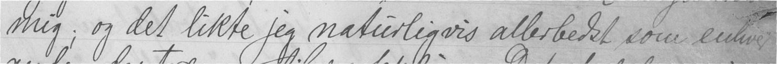mig; og det likte jeg naturligvis allerbedst som enhver
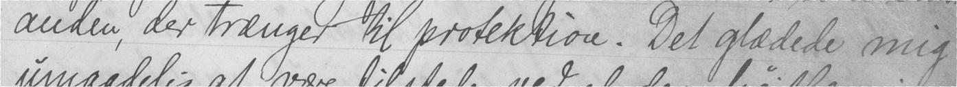anden, der trænger til protektion. Det glædede mig
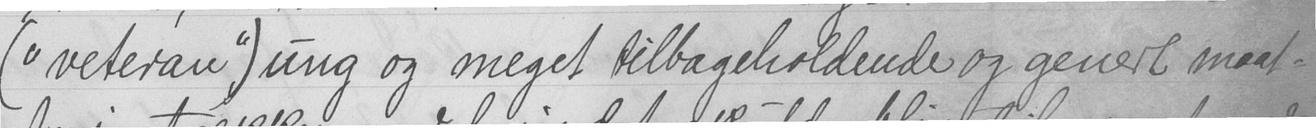("veteran") ung og meget tilbageholdende og genert maat-
